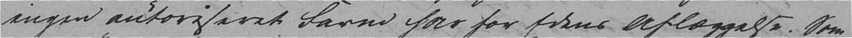ingen autoriseret Form har for Edens aflæggelse. Som
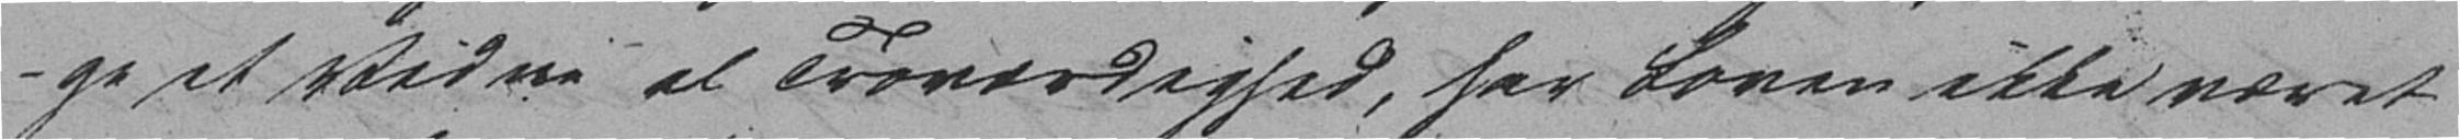ge et Vidne al Troværdighed, har Loven ikke været
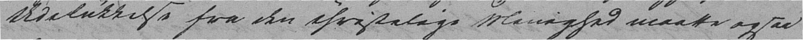udelukkelse fra den christelige Menighed maatte ogsaa
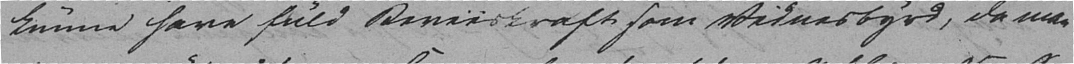kunne have fuld Beviiskraft som Vidnesbyrd, da man
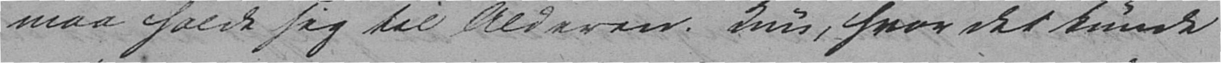maa holde sig til Alderen. Kun, hvor det kunde
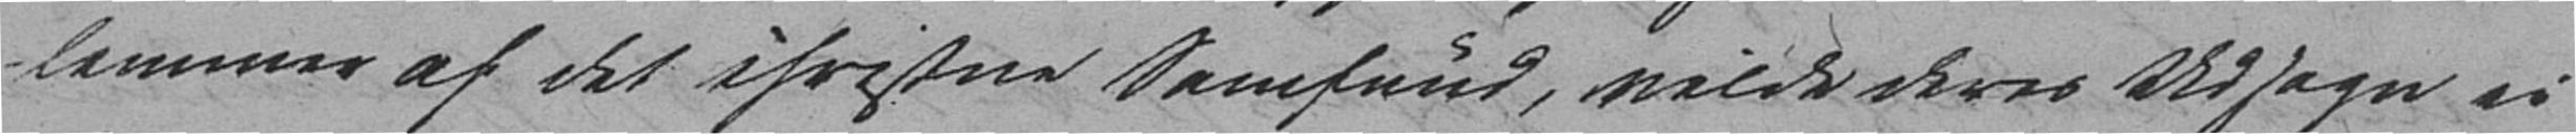lemmer af det christne Samfund, vilde deres Udsagn ei
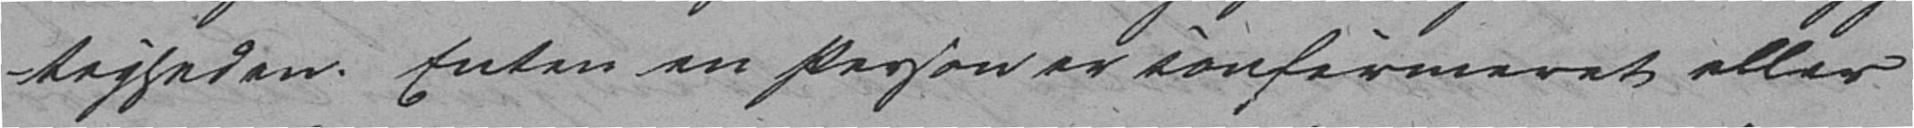tigheden. Enten en Person er confirmeret eller
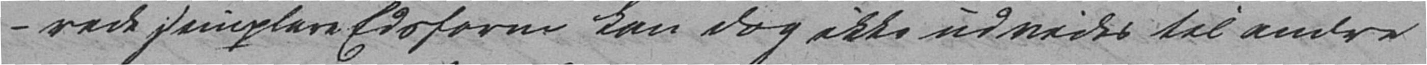rede simplere Edsform kan dog ikke udvides til andre
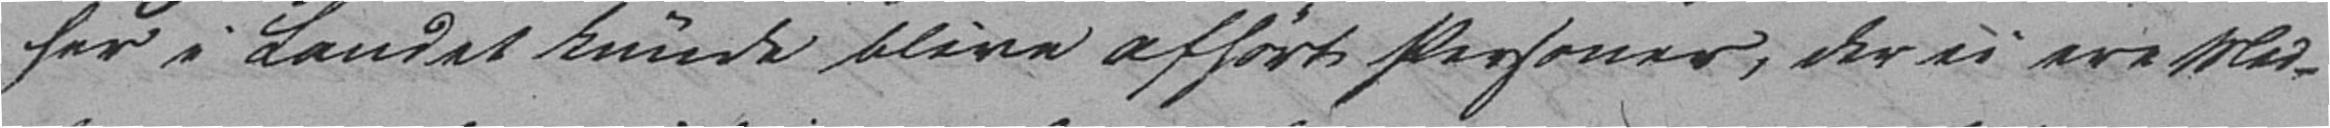her i Landet kunde blive afhørt Personer, der ei ere Med-
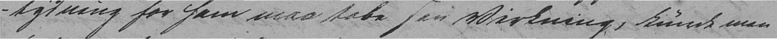tydning for ham maa tabe sin Virkning, kunde man
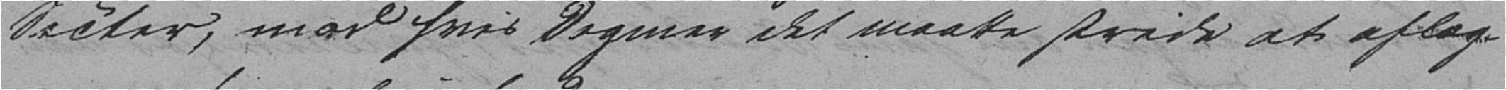Secter, mod hvis Dogmer det maatte stride at aflæg-
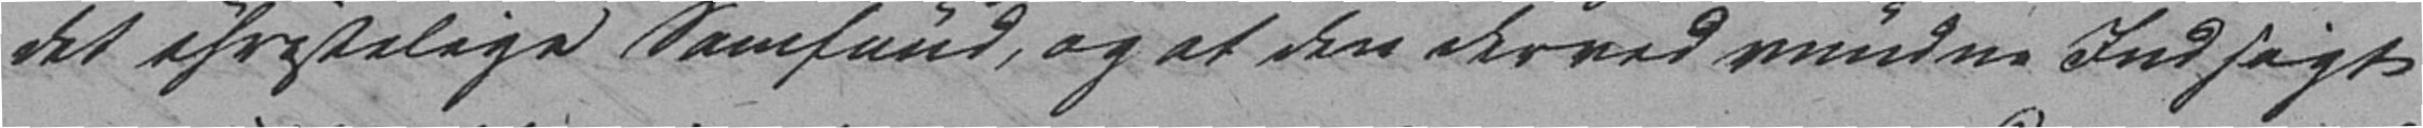det christelige Samfund, og at den derved mindre Indsigt
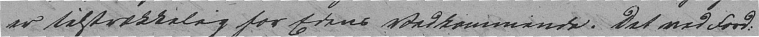er tilstrækkelig for Edens Vedkommende. Det ved Ford.
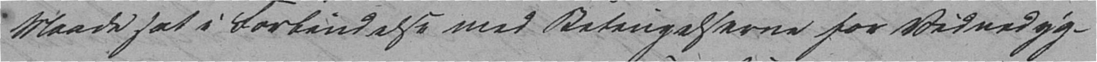Maade sat i Forbindelse med Betingelserne for Vidnedyg-
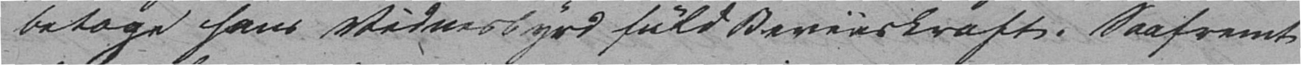betage hans Vidnesbyrd fuld Beviiskraft. Saafremt
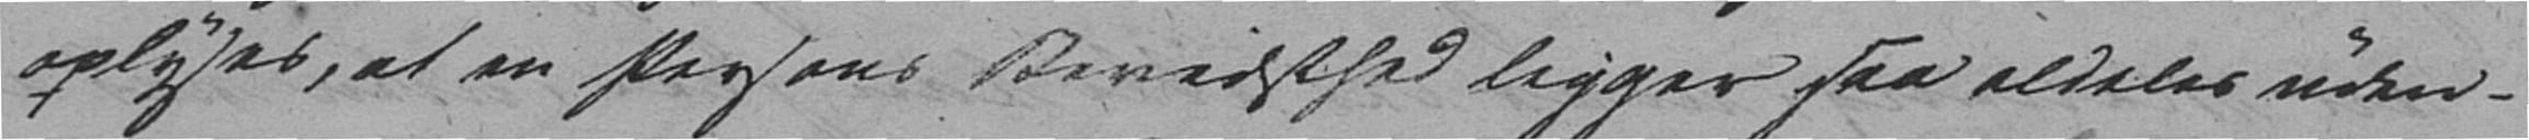oplyses, at en Persons Bevidsthed ligger saa aldeles uden-
